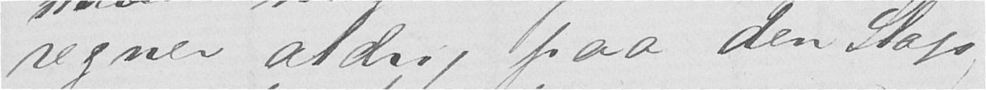regner aldrig paa den Slags
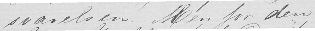svarelsen. Men for den
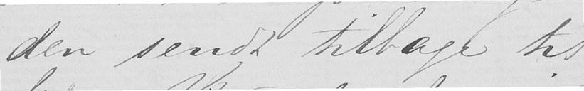den sendt tilbage til
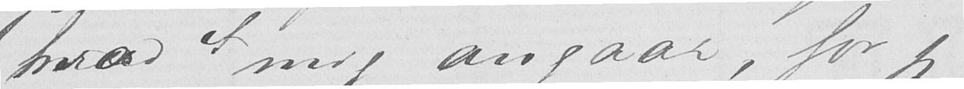hvad mig angaar, for jeg
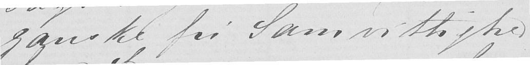ganske fri Samvittighed
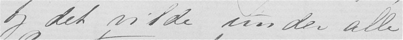og det vilde, under alle
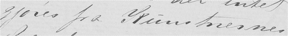gjøres fra Kunstnernes
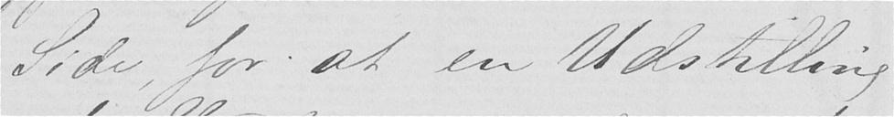Side, for at en Udstilling
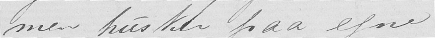men husker paa egne
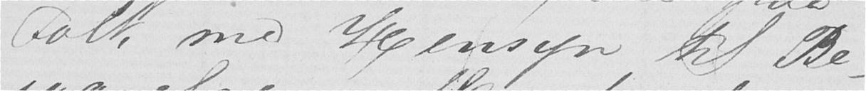Folk med Hensyn til Be-
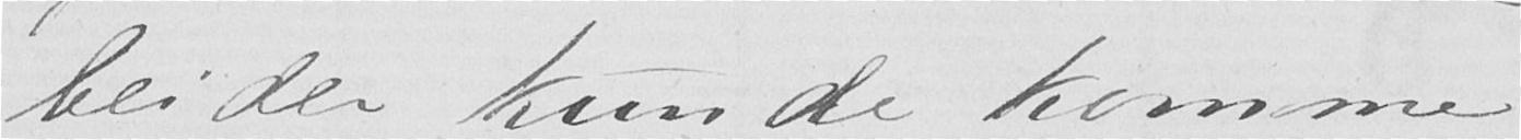beider kunde komme
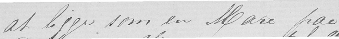at ligge som en Mare paa
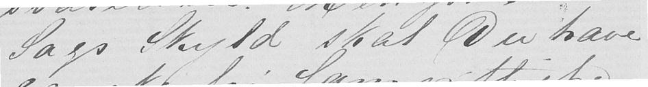Sags Skyld skal Du have
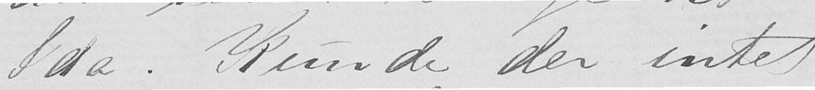Ida. Kunde der intet
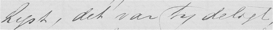Lyst, det var tydeligt
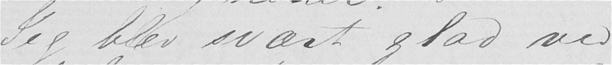Jeg blev svært glad ved
